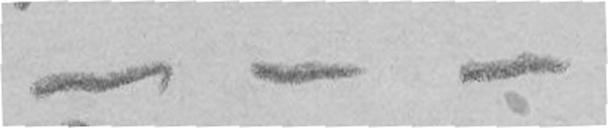- - -
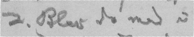2. Blev de med i
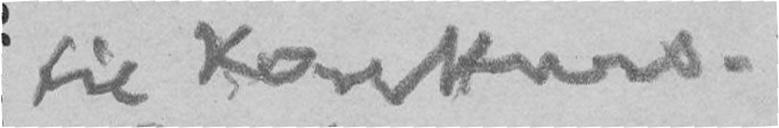til Konkurs.
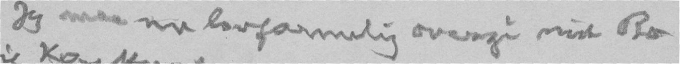Jeg maa nu lovformelig overgi mit Bo
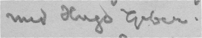med Hugo Geber.
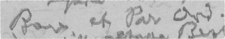Bare et Par Ord.
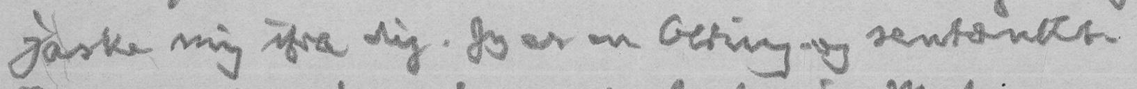jaske mig ifra dig. Jeg er en Olding og sentænkt
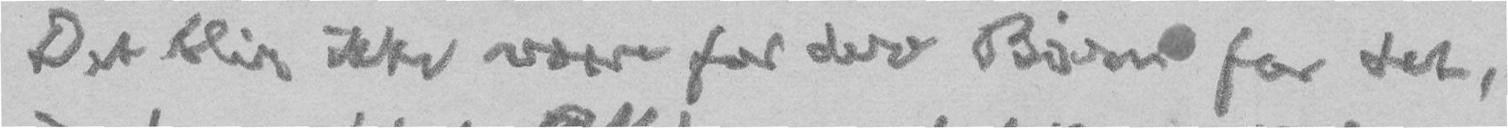Det blir ikke værre for dere Børn for det,
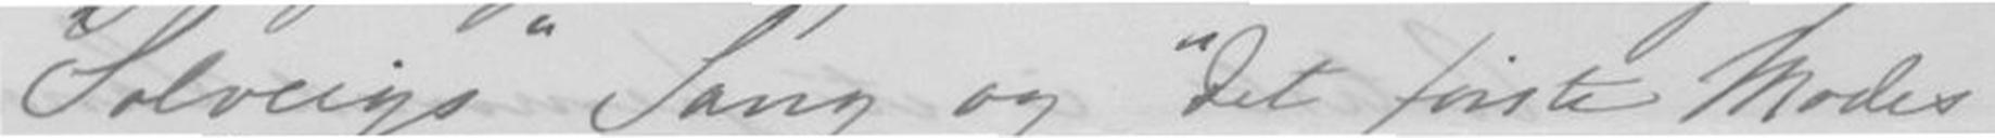"Solveigs" Sang og "Det første Mødes
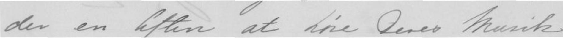der en Aften at høre Deres Musik
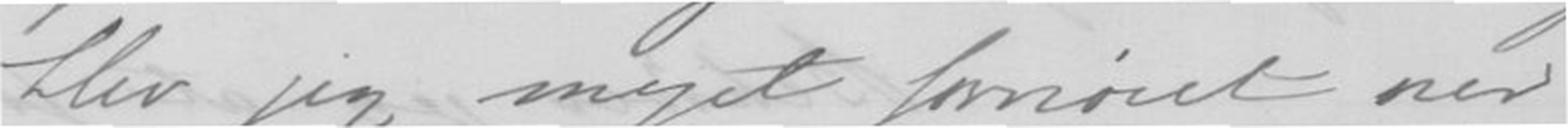blev jeg meget fornøiet med
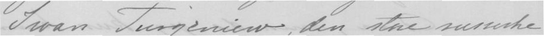Iwan Turgeniew, den store russiske
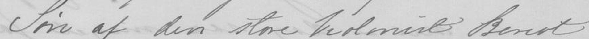Søn af den store Violinist Beriot
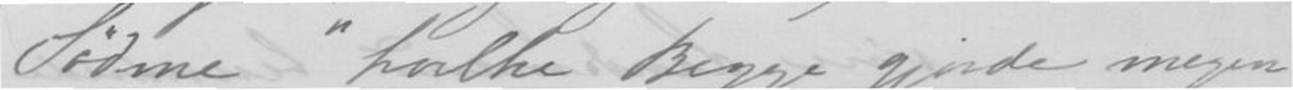Sødme" hvilke begge gjorde megen
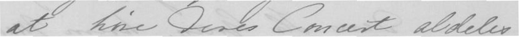at høre Deres Concert aldeles
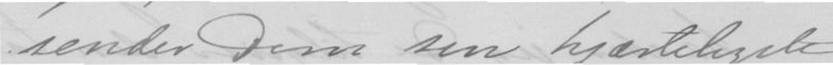sender Dem sin hjærteligste
